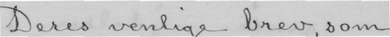Deres venlige brev, som
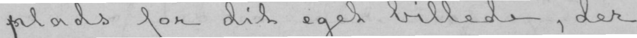splads for dit eget billede, der
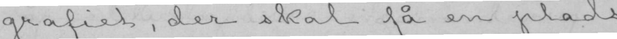grafiet, der skal få en plads
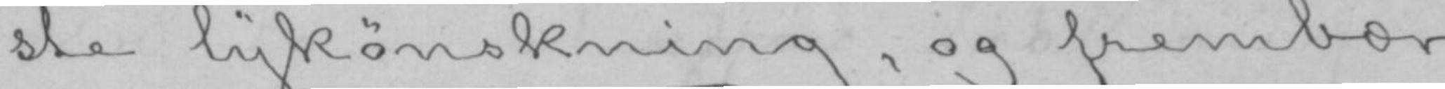ste lykønskning, og frembær
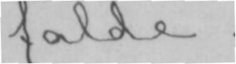falde.
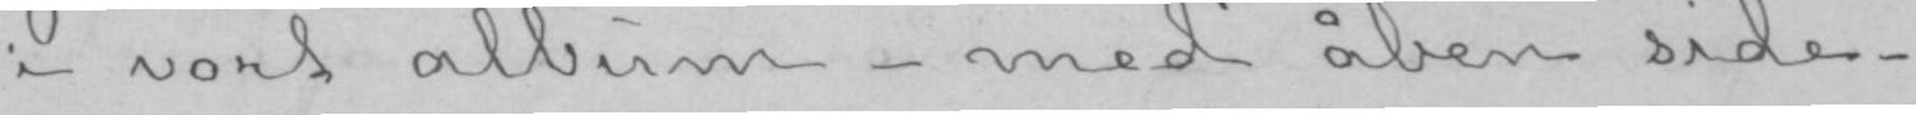i vort album- med åben side-
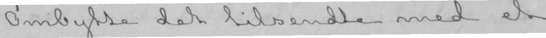Ombytte det tilsendte med et
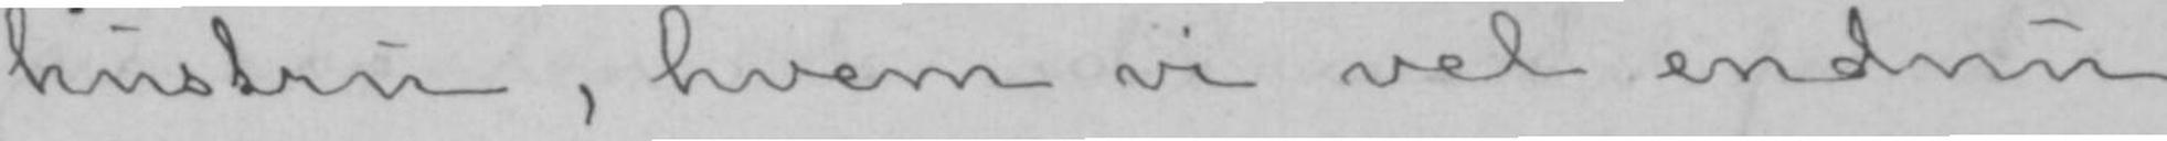hustru, hvem vi vel endnu
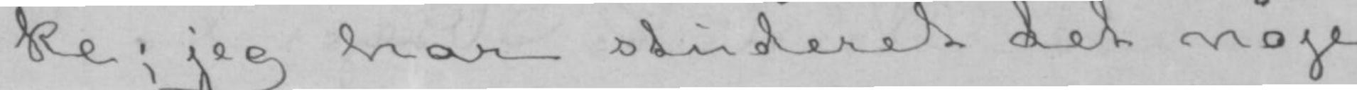ke; jeg har studeret det nøje
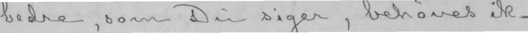bedre, som Du siger, behøves ik-
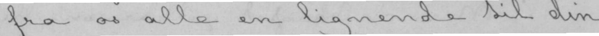fra os alle en lignende til din
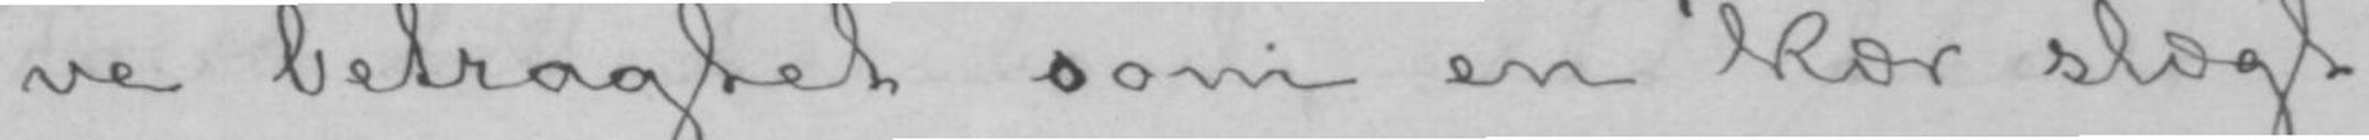ve betragtet som en kær slægt-
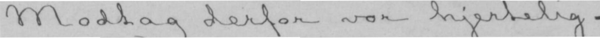Modtag derfor vor hjertelig-
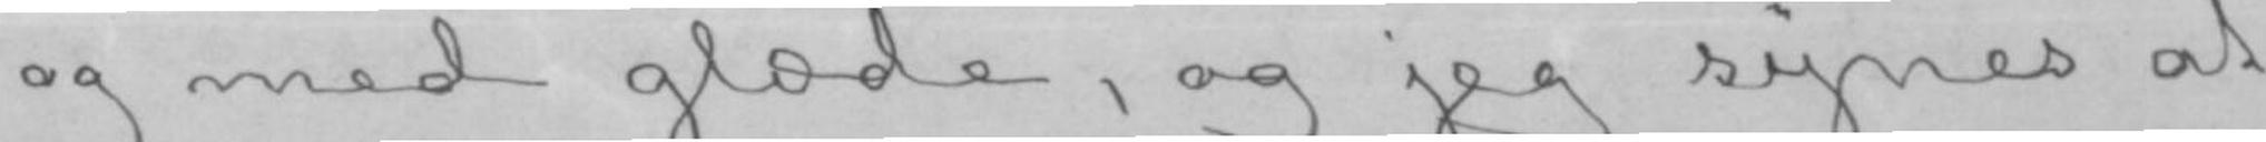og med glæde, og jeg synes at
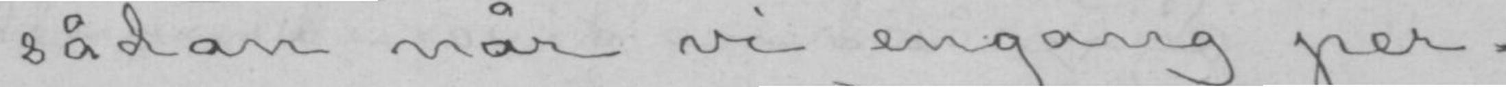sådan når vi engang per-
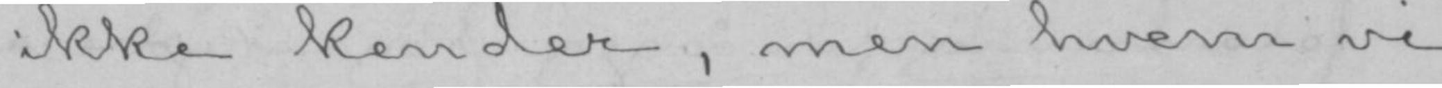ikke kender, men hvem vi
